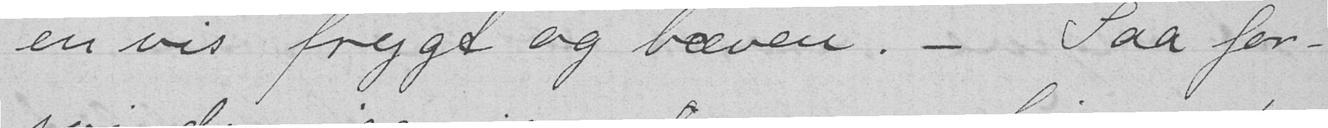en vis frygt og bæven. - Saa for-
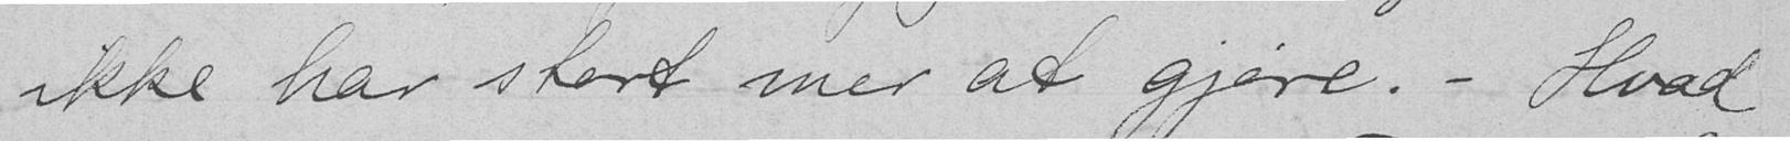ikke har stort mer at gjøre. - Hvad
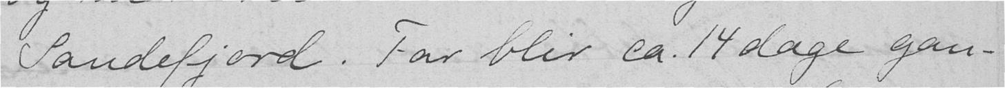Sandefjord. Far blir ca. 14 dage gan-
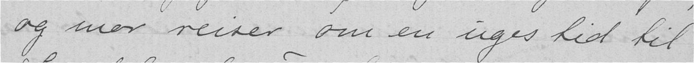og mor reiser om en uges tid til
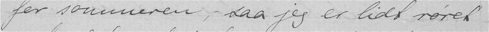før sommeren,- saa jeg er lidt røret
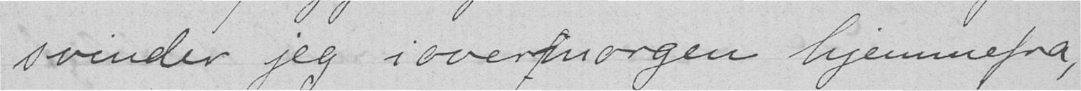svinder jeg ioverimorgen hjemmefra,
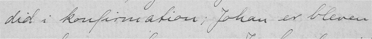did i konfirmation; Johan er bleven
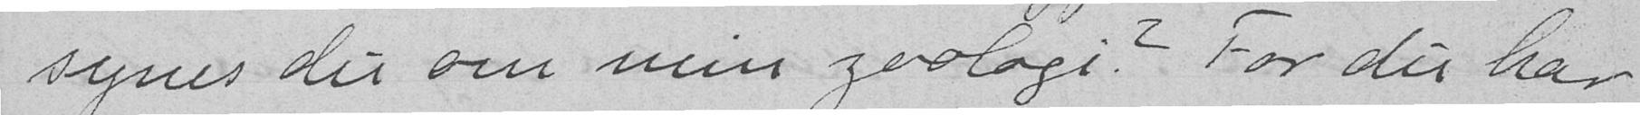synes du om min zoologi? For de har
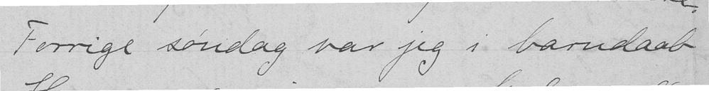Forrige søndag var jeg i barndaab
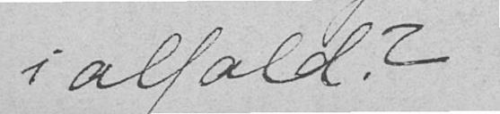i alfald?
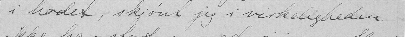i hodet, skjønt jeg i virkeligheden
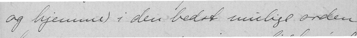og hjemme) i den bedst mulige orden
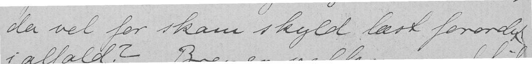da vel for skam skyld læst forordet
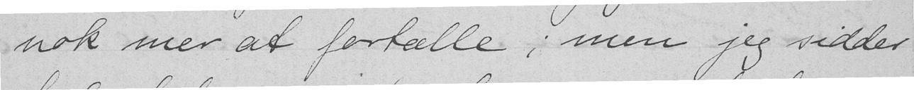nok mer at fortælle; men jeg sidder
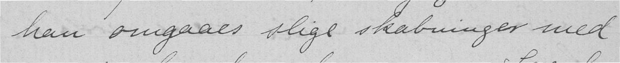han omgaaes slige skabninger med
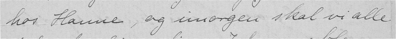hos Hanne, og imorgen skal vi alle
